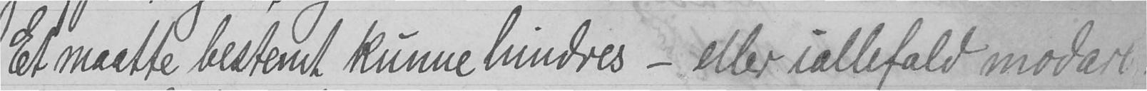Et maatte bestemt kunne hindres - eller iallefald modar
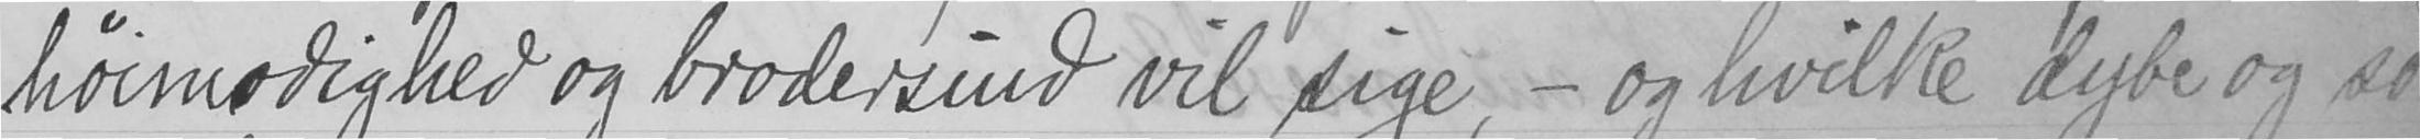høimodighed og brodersind vil sige, - og hvilke dybe og so
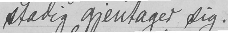stadig gjentager sig.
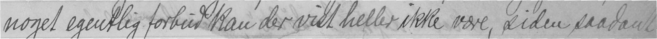noget egentlig forbud kan der vist heller ikke være, siden saadant
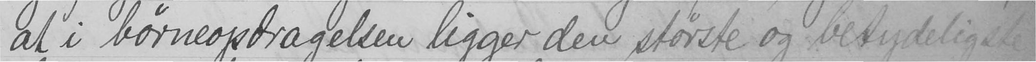at i børneopdragelsen ligger den største og betydeligste
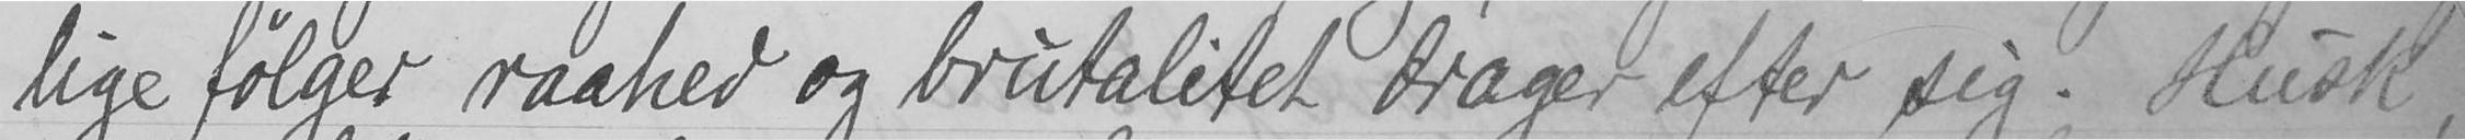lige følger raahed og brutalitet drager efter sig. Husk,
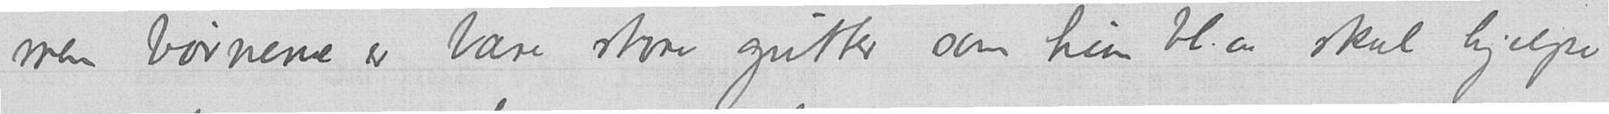men børnene er bare store gutter som hun bl.a. skal hjelpe
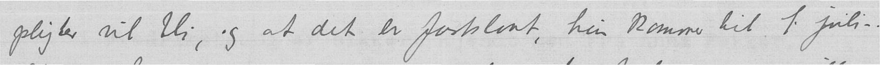pligter vil bli, og at det er fastslaat, hun kommer til 1. juli –.
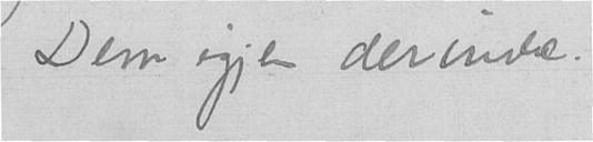Dem igjen derinde.
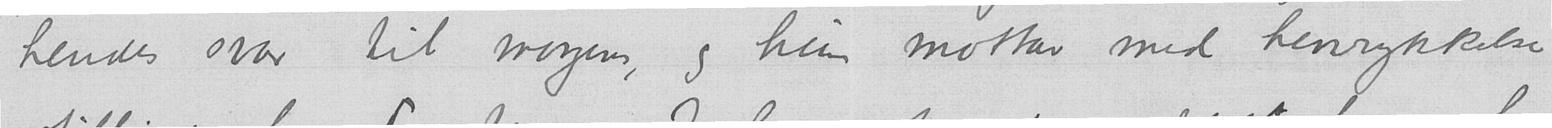hendes svar til morgens, og hun mottar med henrykkelse
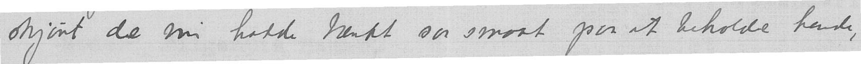skjønt de nu hadde tænkt saa smaat paa at beholde hende,
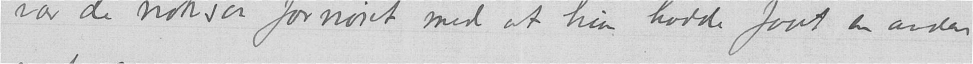var de noksaa fornøiet med at hun hadde faat en anden
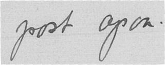post ogsaa.
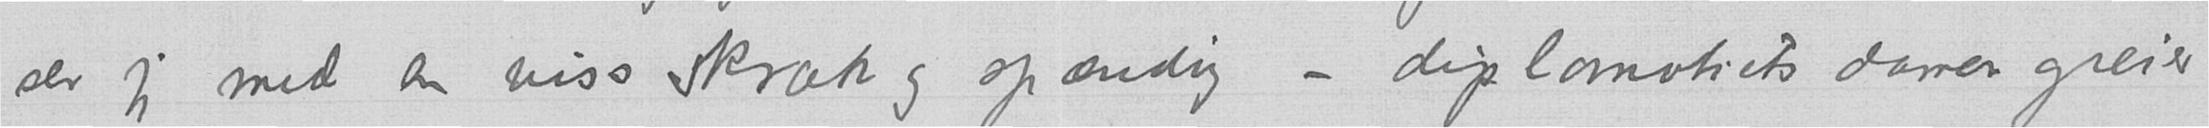ser jeg med en viss skræk og spænding – diplomatiets damer greier
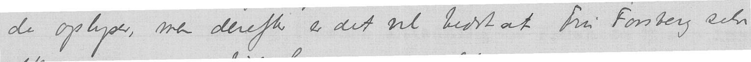de oplyser, men derefter er det vel bedst at Fru Forsberg selv
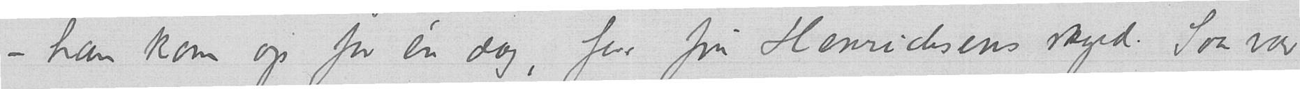– han kom op for en dag, for fru Henrichsens skyld. Saa var
